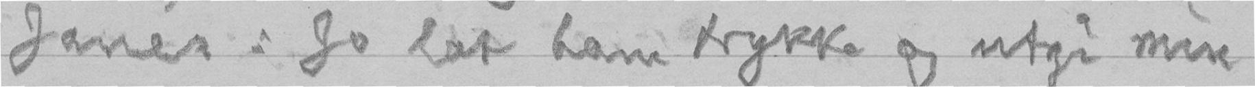Janés: Jo lat ham trykke og utgi min
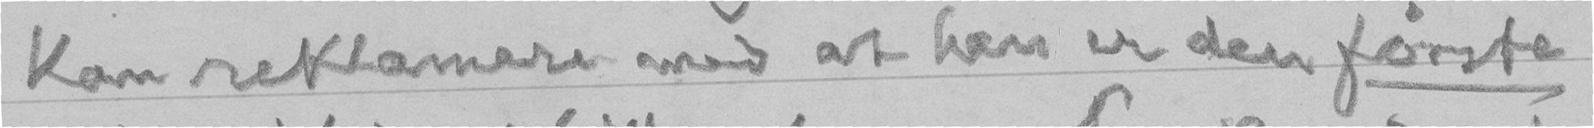kan reklamere med at han er den første
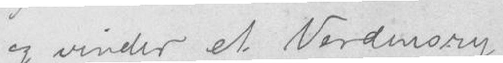og vinder et Verdensry -
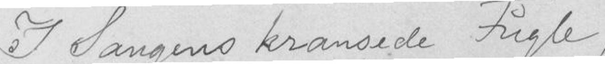I Sangens kransede Fugle,
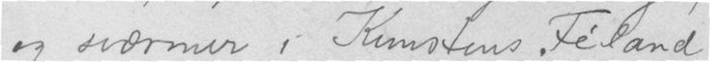og nærmer i Kunstens Fèland,
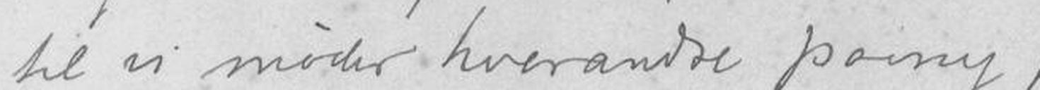til vi møder hverandre paany,
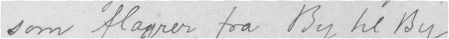som flagrer fra By til By,
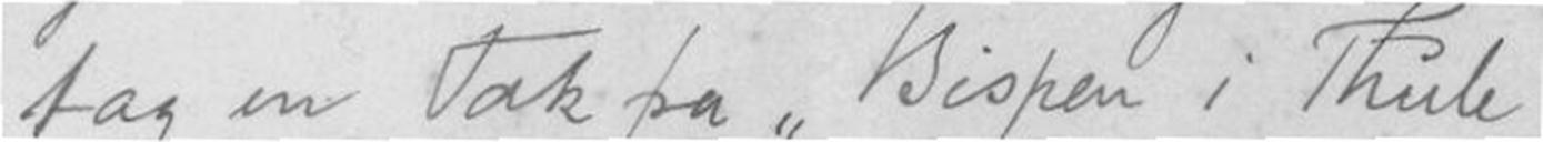tag en Tak fra "Bispen i Thule!,
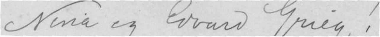Nina og Edvard Grieg!
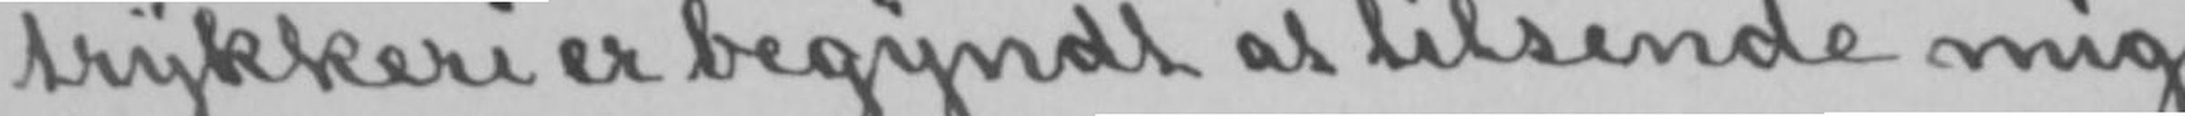trykkeri er begyndt at tilsende mig
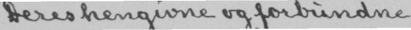Deres hengivne og forbundne
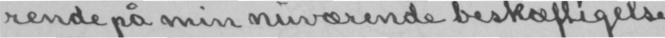rende på min nuværende beskæftigelse
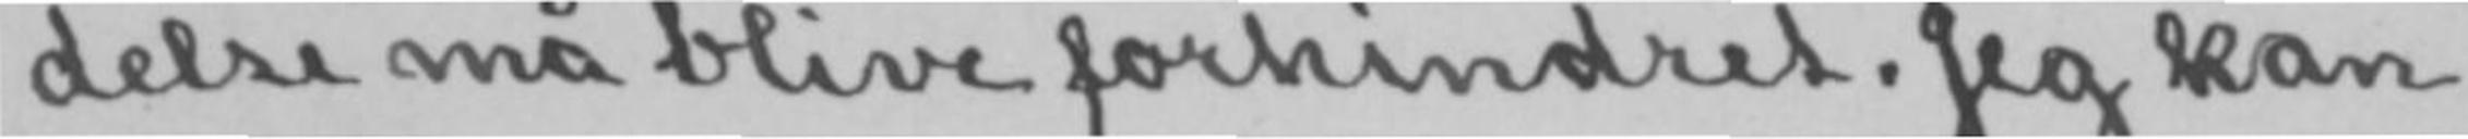delse må blive forhindret. Jeg kan
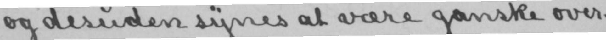og desuden synes at være ganske over-
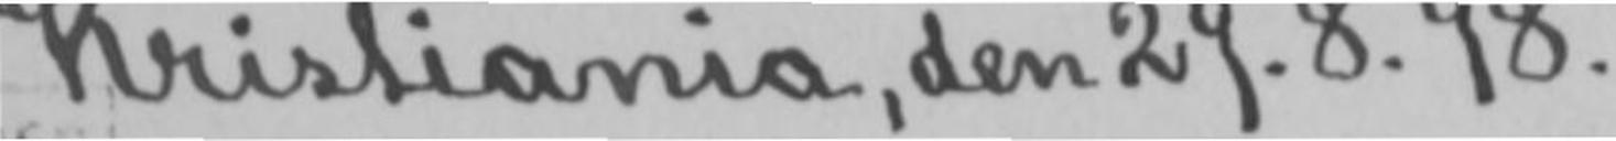Kristiania, den 29.8.98.
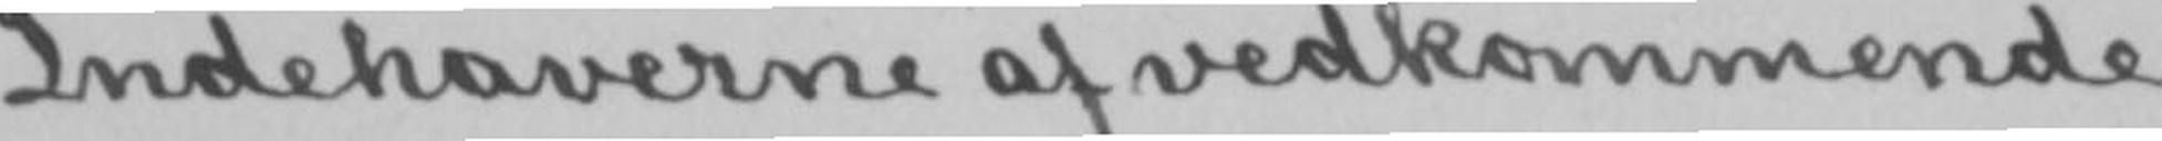Indehaverne af vedkommende
